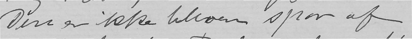Den er ikke bleven spor af
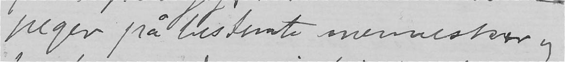peger på bestemte mennesker og
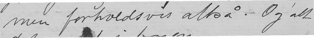men forholdsvis altså. - Og alt
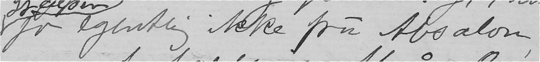jo egentlig ikke fru Absalon,
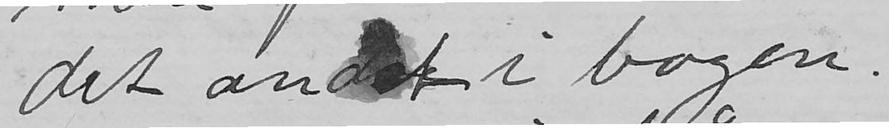det andet i bogen.
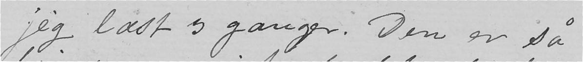jeg læst 3 ganger. Den er så
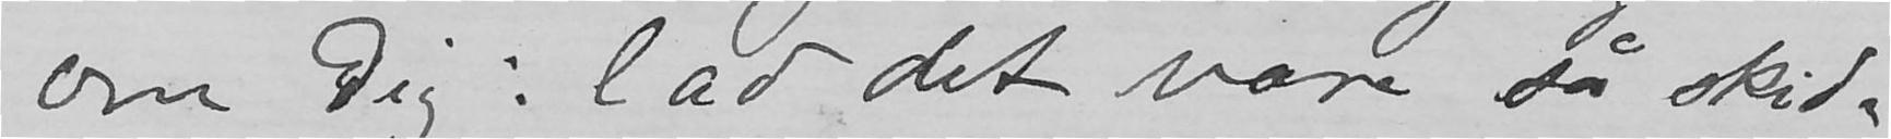om Dig: lad det være så skid-
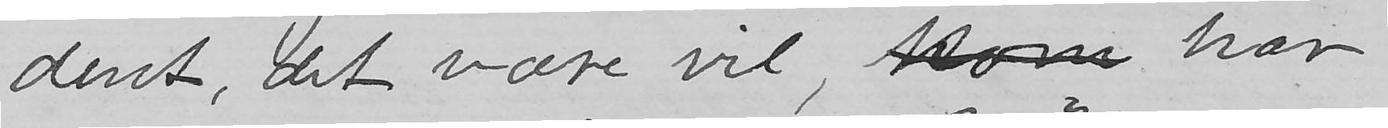dent, det være vil, kom har
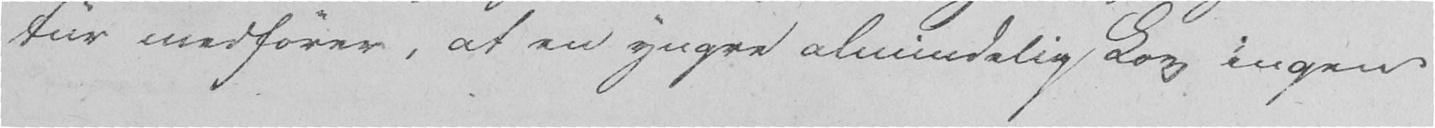tur medfører, at en yngre almindelig Lov ingen
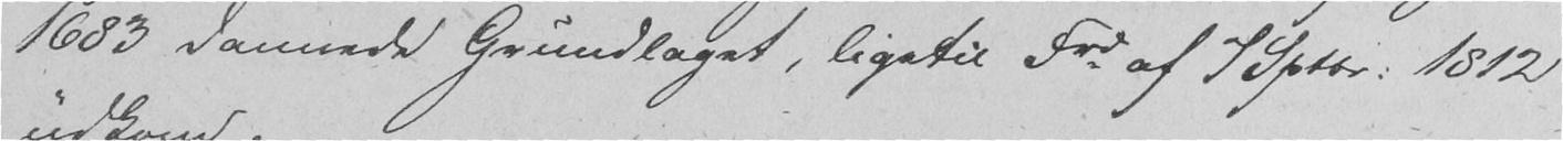1683 dannede Grundlaget, ligetil Frd af 7 Sptbr. 1812
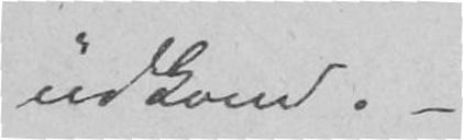udkom. -
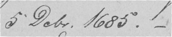5 Dcbr. 1685. -
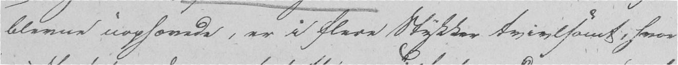blevne uophævede, er i flere Stykker tvivlsomt, hvor
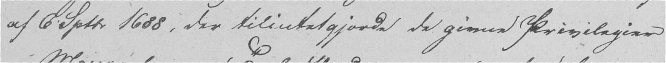af 6 Sptbr 1688, der tilintetgjorde de givne Privilegier
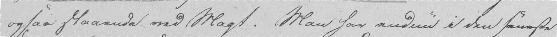ogsaa staaende ved Magt. Man har endnu i den seneste
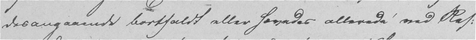desangaaende bortfaldt eller hævedes allerede ved Res.
